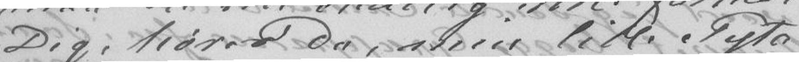Dig, hører Du, min lille Tyta?
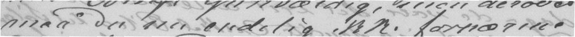maa Du nu endelig ikke fornærme
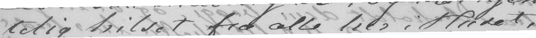telig hilset fra alle her i Huset,
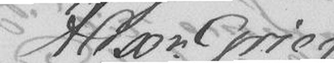Alex r Grieg
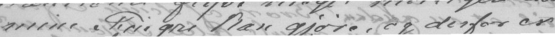mine Fingre kan gjøre, og derfor er
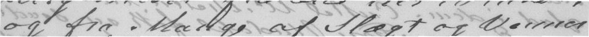og fra Mange af Slægt og Venner
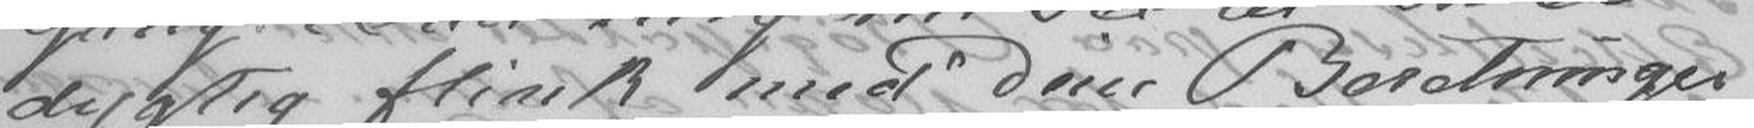dygtig flink med Dine Beretninger
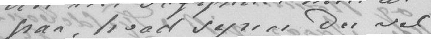paa, hvad syner Du vel
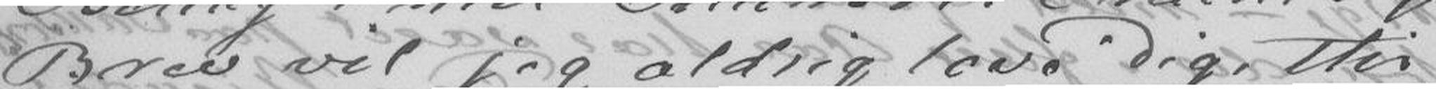Brev vil jeg aldrig love Dig, thi
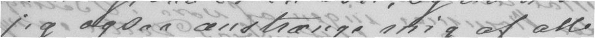jeg ogsaa anstrænge mig af alle
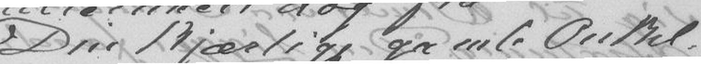Din kjærlige gamle Onkel
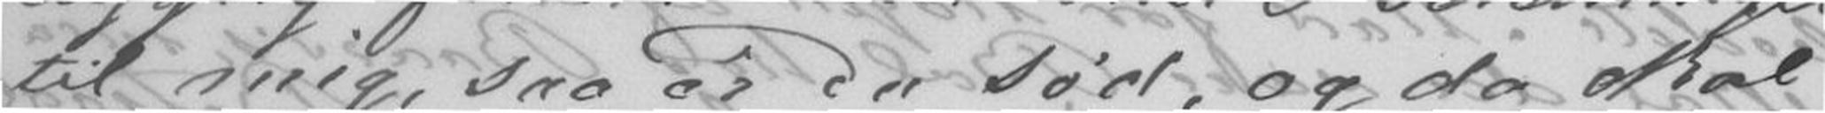til mig, saa er Du sød, og da skal
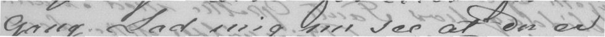Gang. Lad mig nu see at Du er
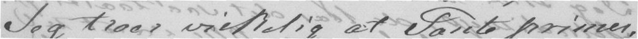Jeg troer virkelig at Tante primer,
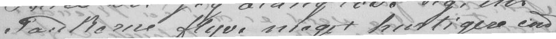Tankerne flyve meget hurtigere end
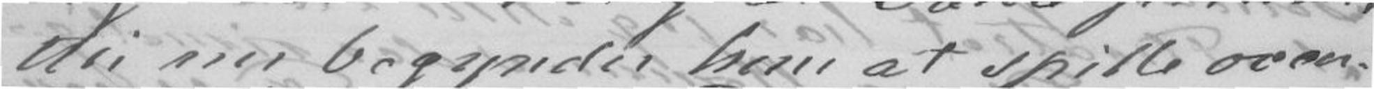thi nu begynder hun at spille oven-
